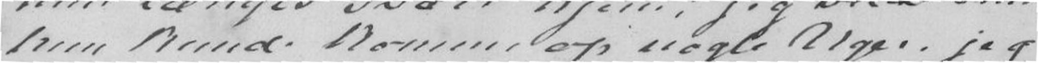hun kunde komme op nogle Uger, jeg
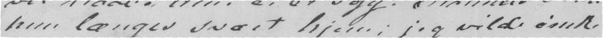hun længes svært hjem; jeg vilde ønske
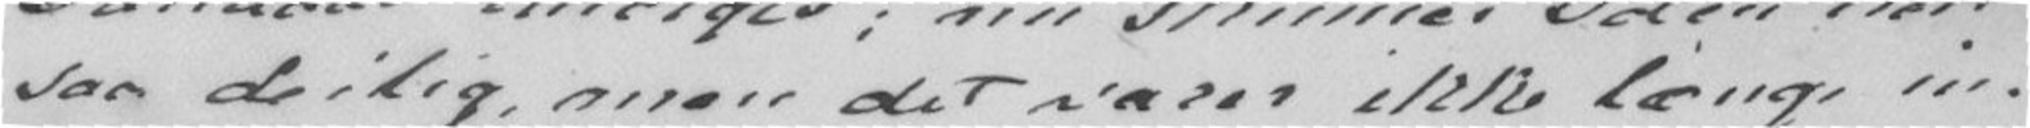saa deilig, men det varer ikke længe in-
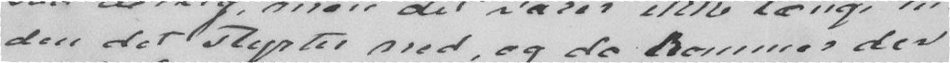den det styrter ned, og da kommer der
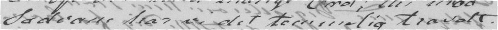sædvane har vi det temmelig travelt
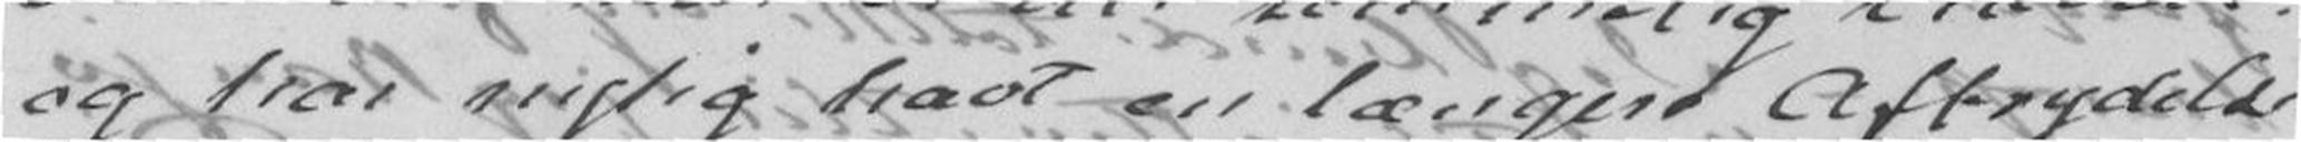og har nylig havt en længere Afbrytelse
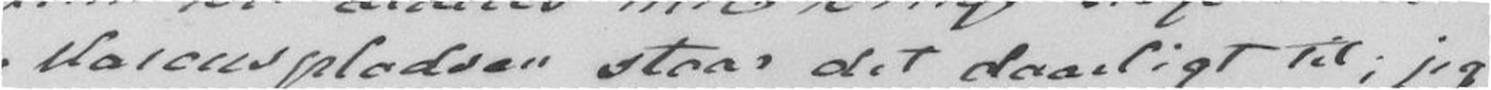Marcuspladsen staar det daarligt til; jeg
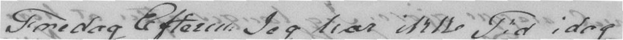Fredag Efterm. Jeg har ikke Tid idag
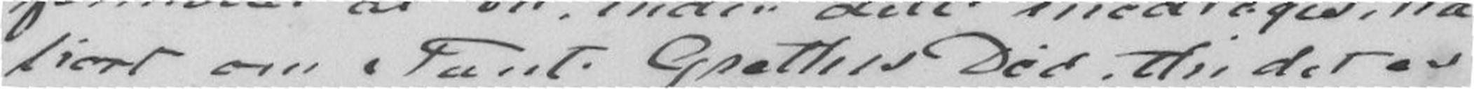hørt om Tante Grethes Død, thi det er
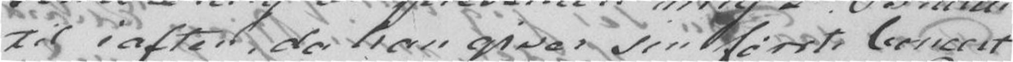til iaften, da han giver sin første Concert
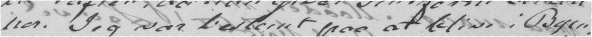her. Jeg var bestemt paa at blive i Byen
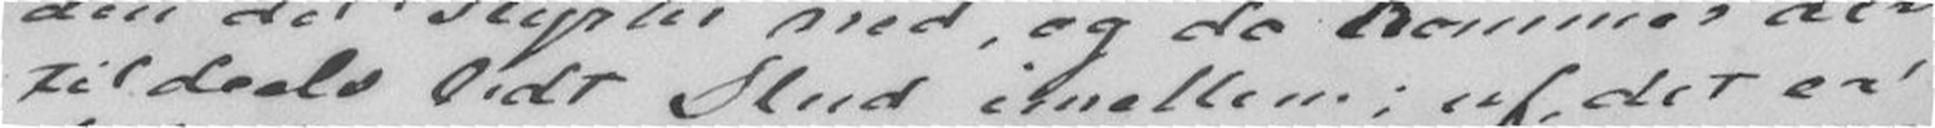tildeels lidt Slud imellem; uf, det er
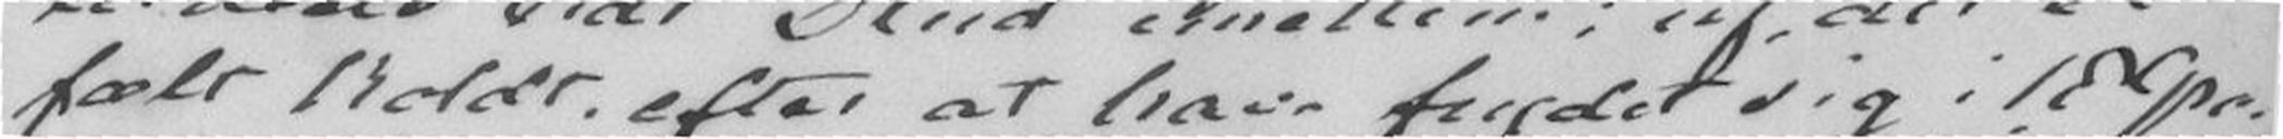fælt koldt efter at have frydet sig i 18 Gr-
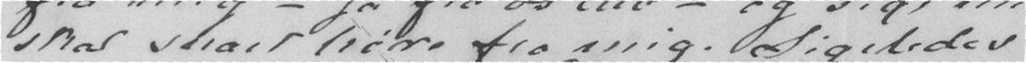skal snart høre fra mig. Ligeledes
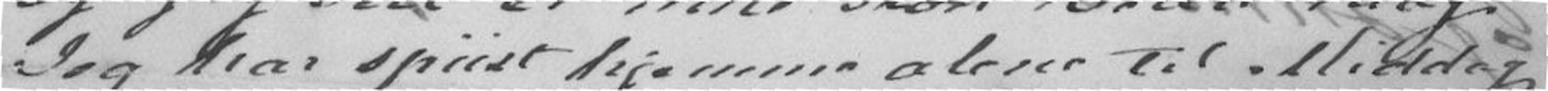Jeg har spiist hjemme alene til Middag
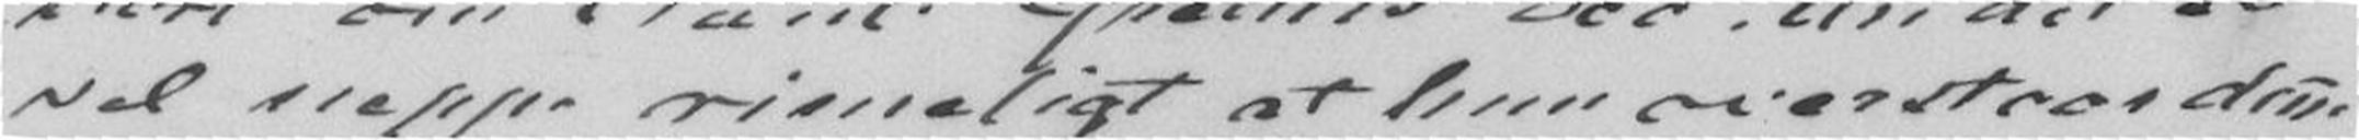vel neppe rimeligt at hun overstaar de`ne
